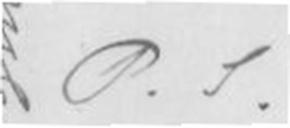P.S.
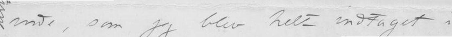inde, som jeg blev helt indtaget
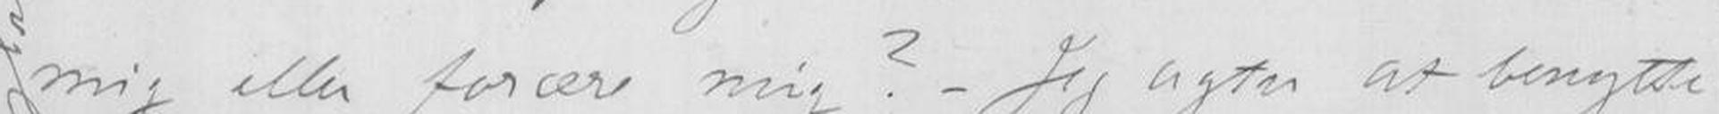mig eller forære mig? - Jeg agter at benytte
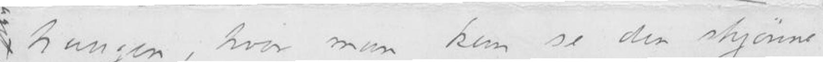haugen, hvor man kan se den skjønne
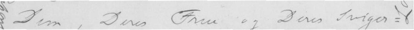Dem, Deres Frue og Deres Sviger-
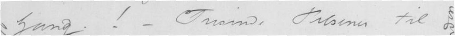Gang! - Tusinde Hilsener til
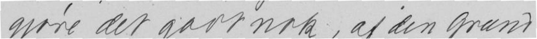gjøre det godt nok, af den Grund
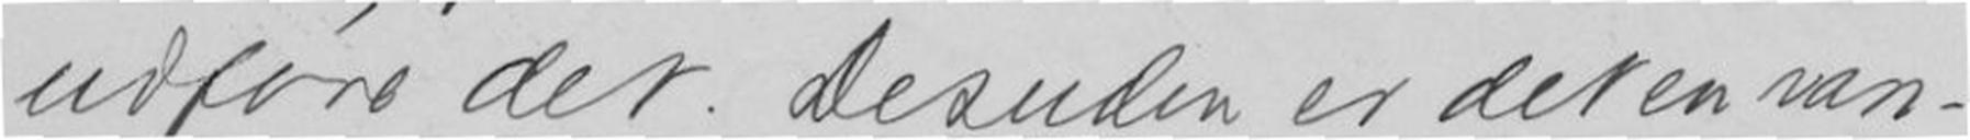udføre det. Desuden er det en van-
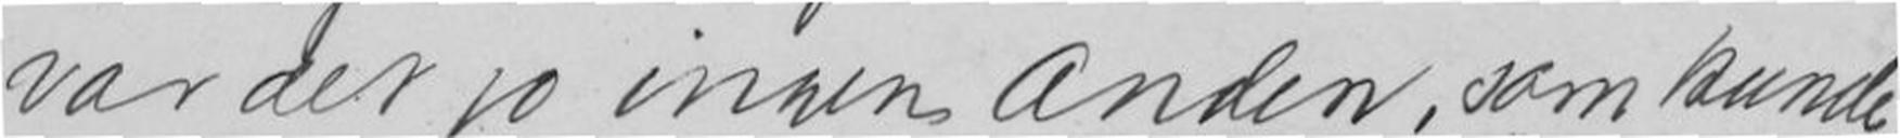var det jo ingen Anden, som kunde
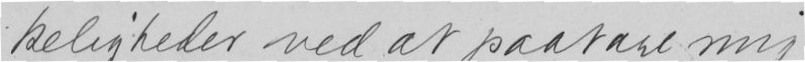keligheder ved at paatage mig
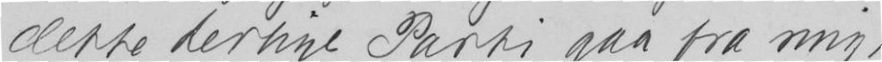dette der Pa gaa fra mig,
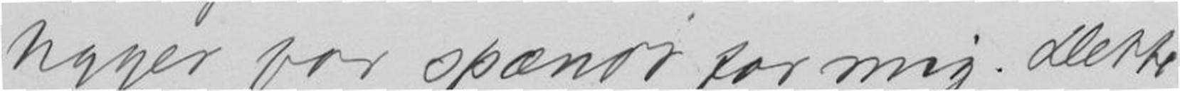saa spændt for mig. Dette
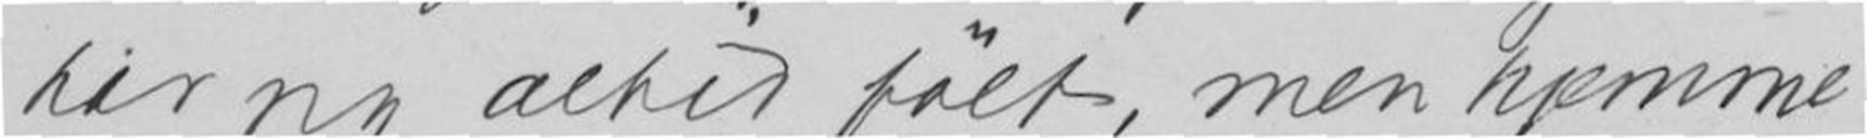har jeg altid følt, men hjemme
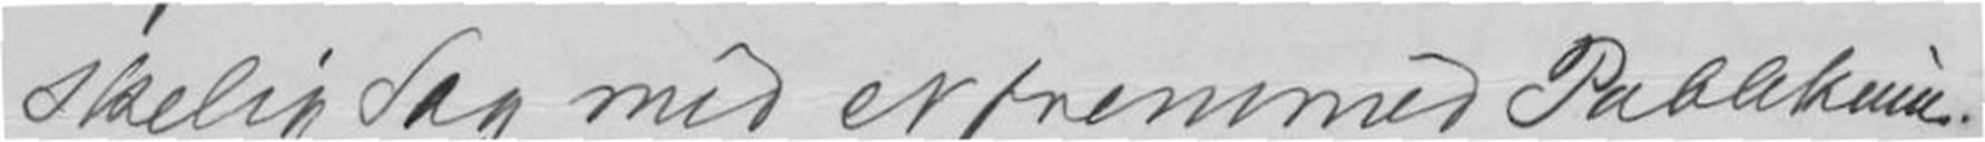skelig Sag med et fremmed Publikum.
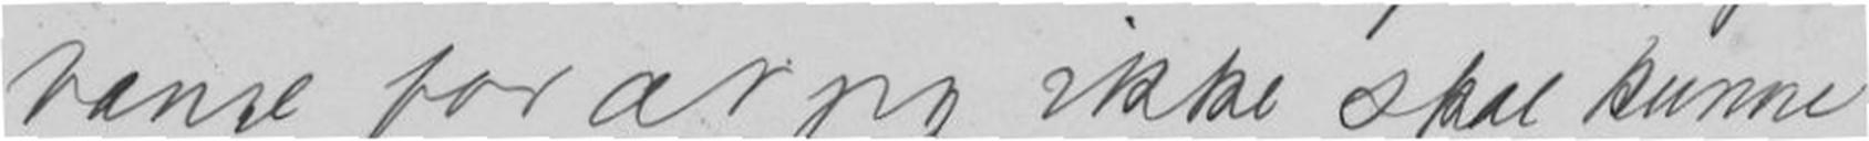for at jeg ikke skal kunne
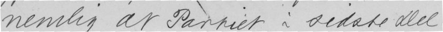nemlig at Partiet i sidste Del
